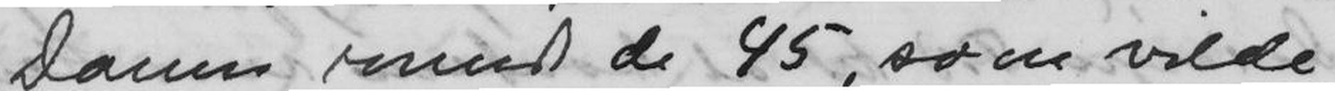Damer rundt de 45, som vilde
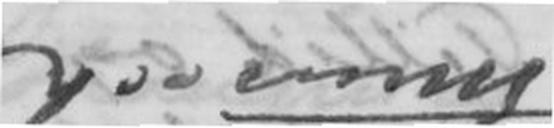hengivne
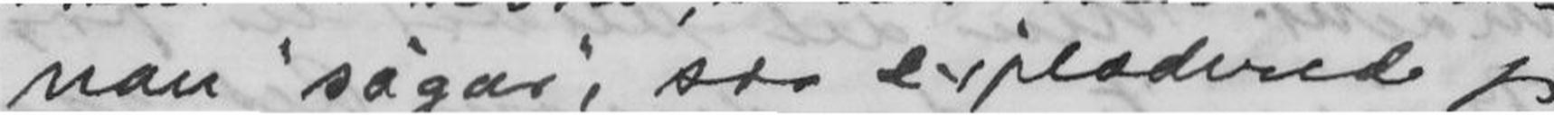nan säger, saa exploderede jeg
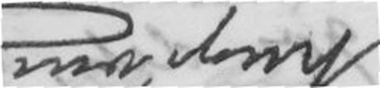Ellen Beck
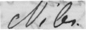Nibr.
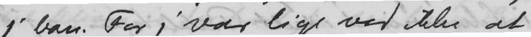j bare. For j var lige ved ikke at
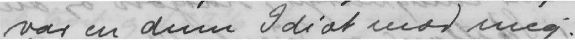var en dum Idiot mod mig.
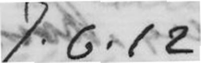7.6.12
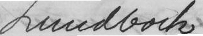Lundbaek
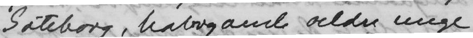Gøteborg, halvgamle aeldre unge
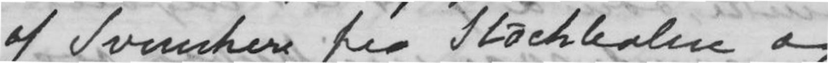af Svenckere fra Stockholm og
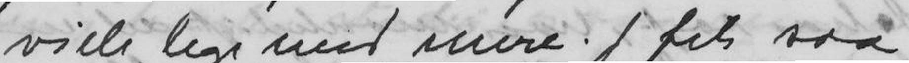ville lege med mere. J fik saa
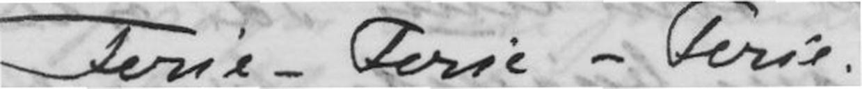Ferie- Ferie - Ferie!
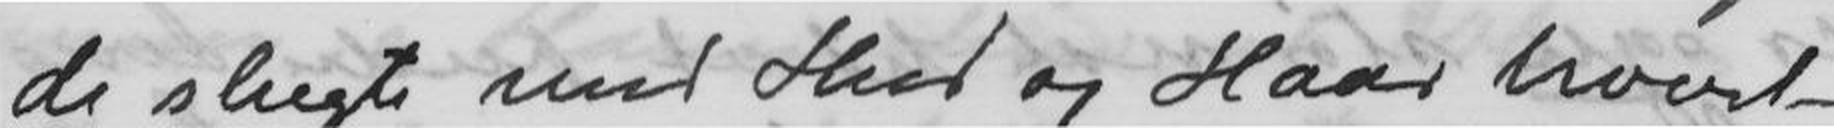de slugte med Hud og Haar hvert
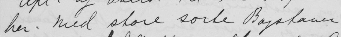her. Med store sorte Bogstaver
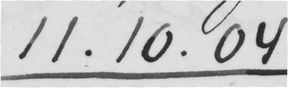11.10.04
